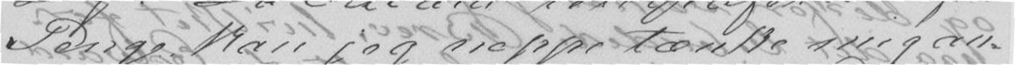Penge kan jeg neppe tænke mig an-
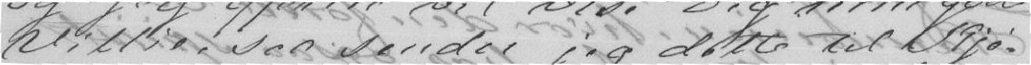Villie saa sender jeg dette til Kjø-
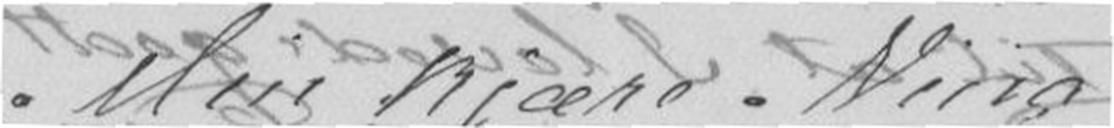Min kjære Nina!
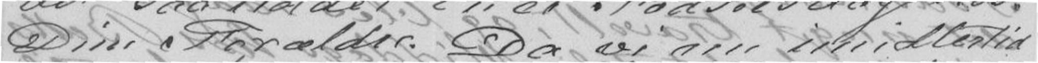Dine Forældre. Da vi nu imidlertid
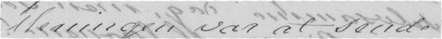Meningen var at sende
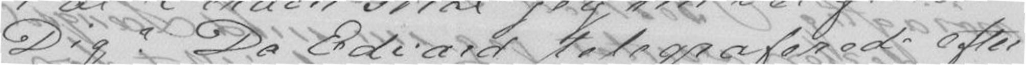Dig? Da Edvard telegraferede efter
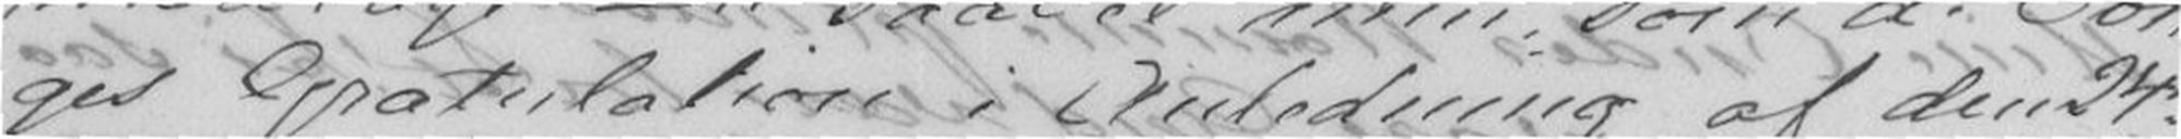ges Gratulation i Anledning af den 24de
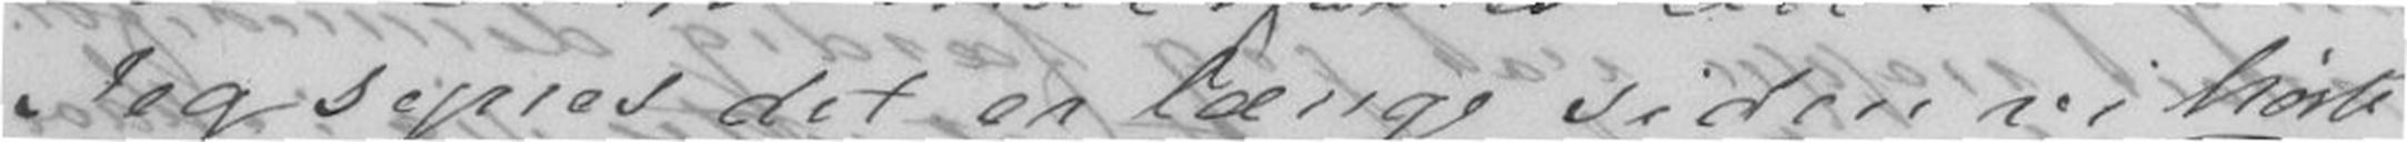Jeg synes det er længe siden vi hørte
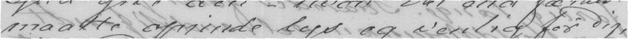maatte oprinde lys og venlig for Dig,
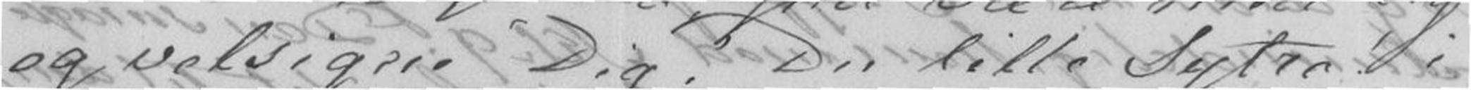og velsigne Dig. Du lille Sytre! i
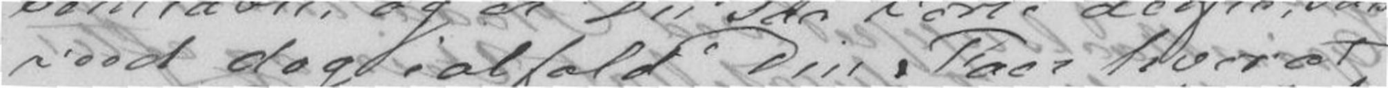veed dog ialfald Din Faer hvorat
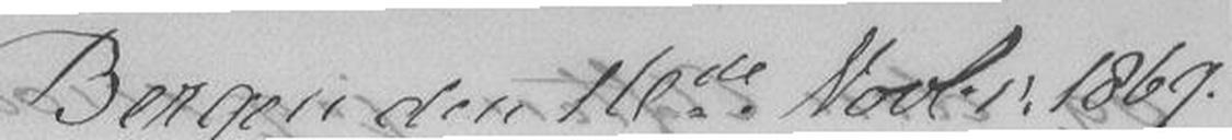Bergen den 16de Novbr. 1869.
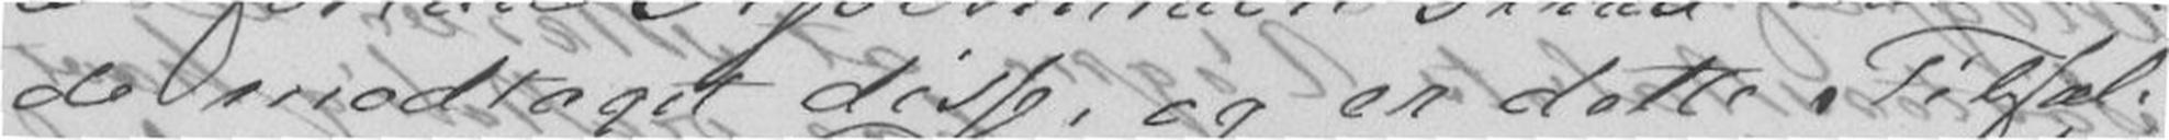de modtaget disse, og er dette Tilfæl-
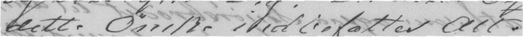dette Ønske indbefattes Alt.
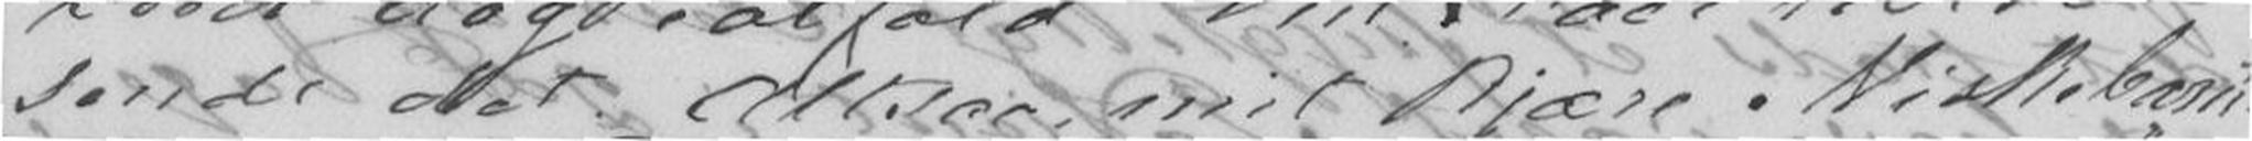sende det. Altsaa mit kjære Niskebarn
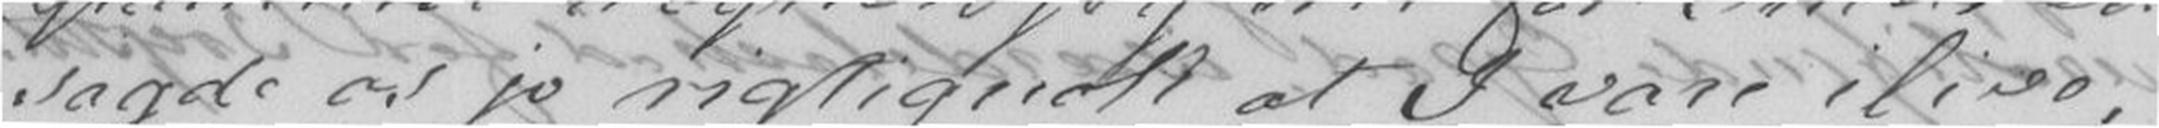sagde os jo rigtignok at I vare ilive,
